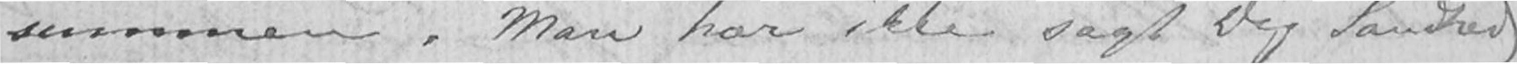summen. Man har ikke sagt Dig Sandhed
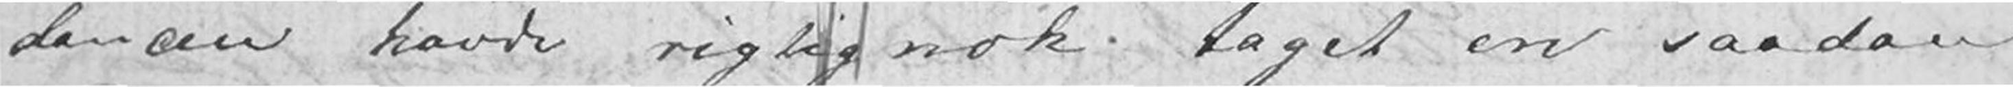dancen havde rigtignok taget en saadan
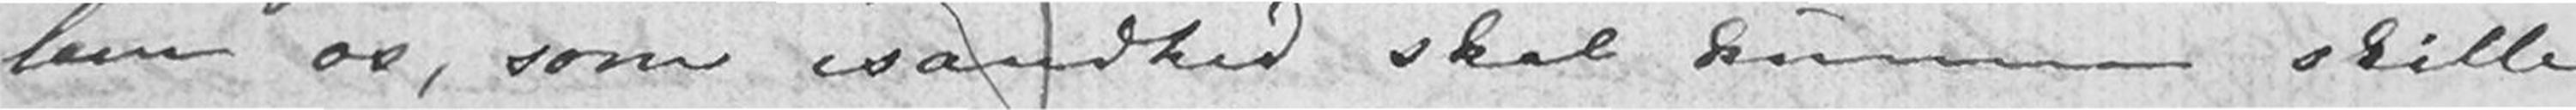lem os, som isandhed skal kunne skille
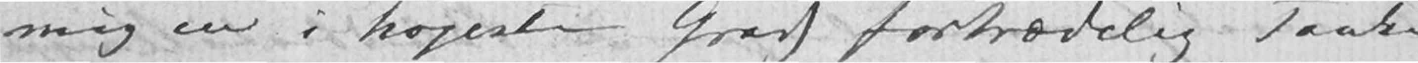mig en i højeste Grad fortrædelig Tanke,
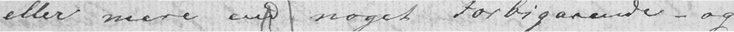eller mere end noget Forbigaaende - og
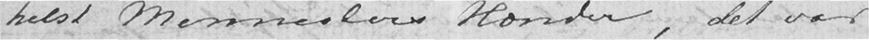helst Menneskers Hænder, det var
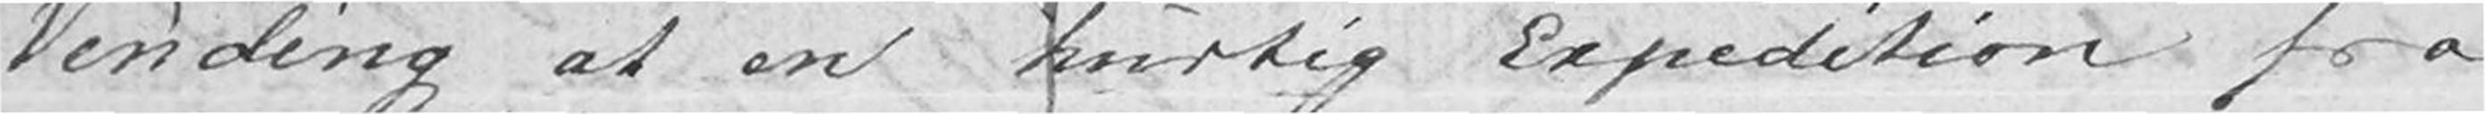Vending at en hurtig Expedition fra
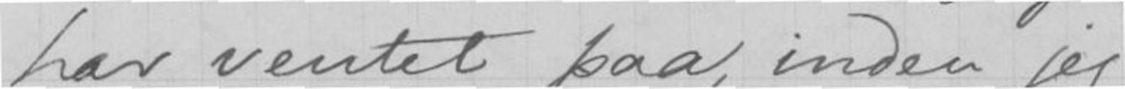har ventet paa, inden jeg
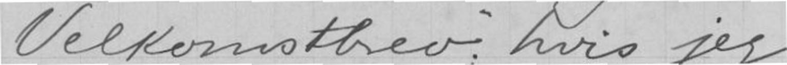Velkomstbrev; hvis jeg
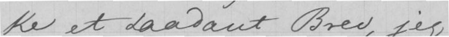ke et saadant Brev, jeg
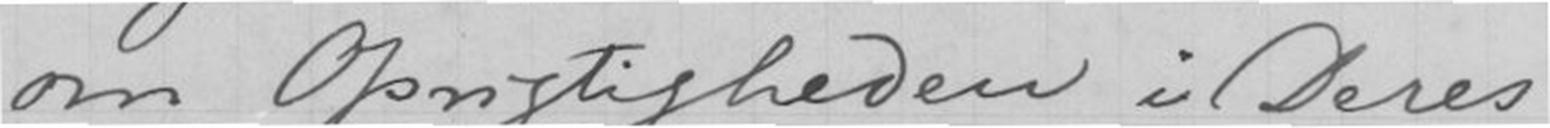om Oprigtigheden i Deres
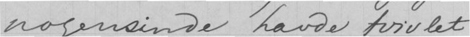nogensinde havde tvivlet
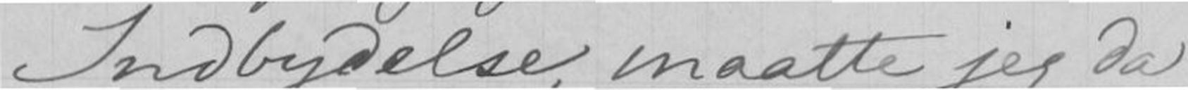Indbydelse, maatte jeg da
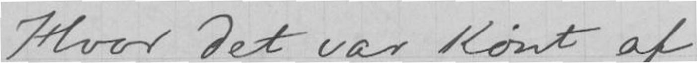Hvor det var Kønt af
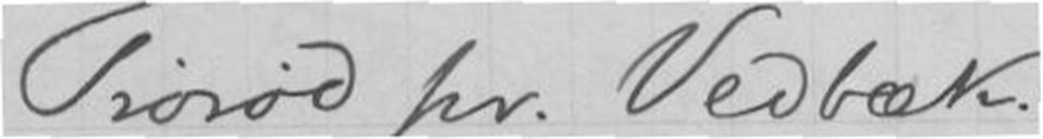Trørød pr. Vedbæk.
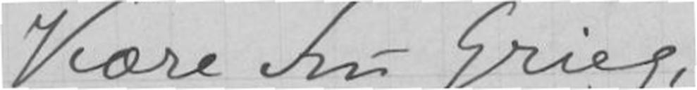Kære fru Grieg,
